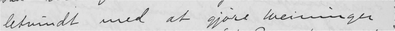letvindt med at gjøre Weininger
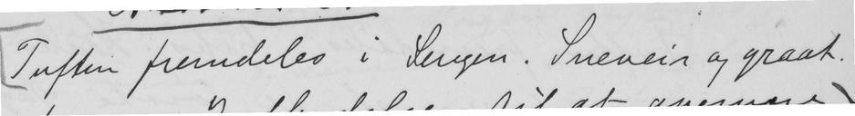Tuften fremdeles i Sengen. Sneveir og graat.
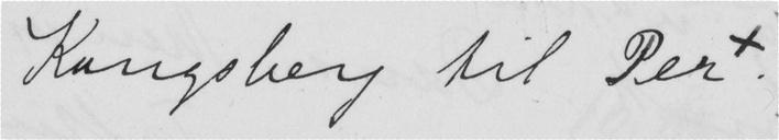Kongsberg til Per. x
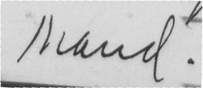Mand
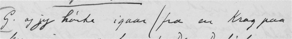G. og jeg hørte igaar (fra en Krog paa
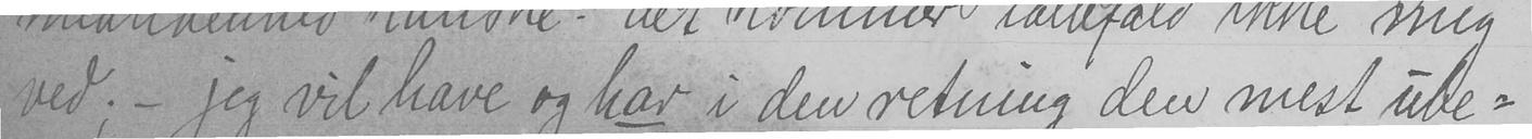ved; - jeg vil have og har i den retning den mest ube-
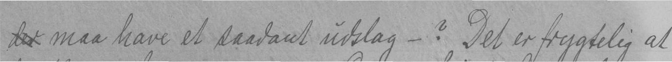der maa have et saadant udslag - ? Det er frygtelig at
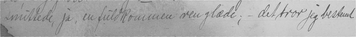smittede, ja, en fuldkommen ren glæde; - det tror jeg bestemt
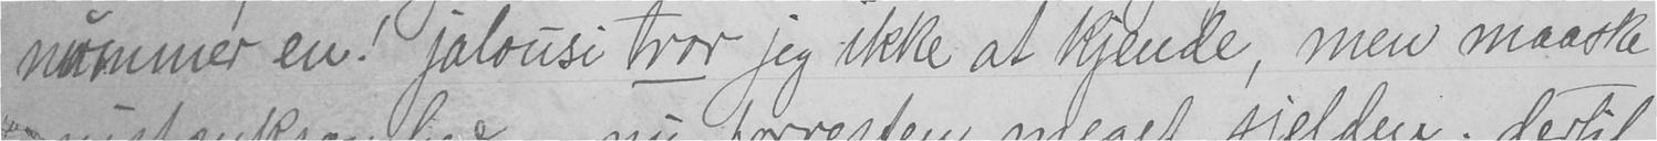nummer en! jalousi tror jeg ikke at kjende, men maaske
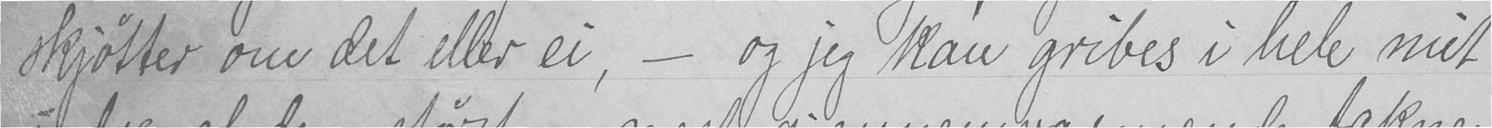skjøtter om det eller ei, - og jeg kan gribes i hele mit
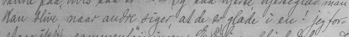kan blive naar andre siger, at de er glade i en! Jeg for-
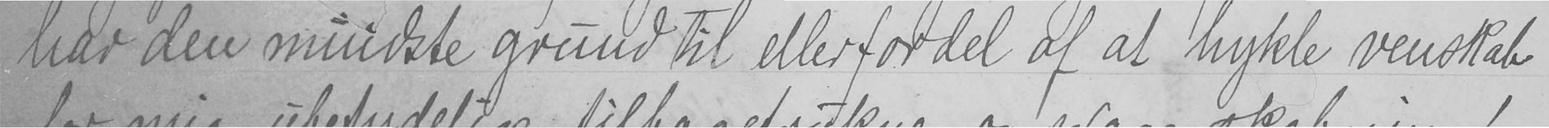har den mindste grund til eller fordel af at hykle venskab
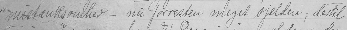mistænksomhed - nu forresten meget sjælden; dertil
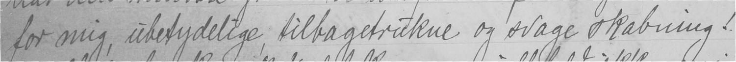for mig, ubetydelige, tilbagetrukne og svage skabning!
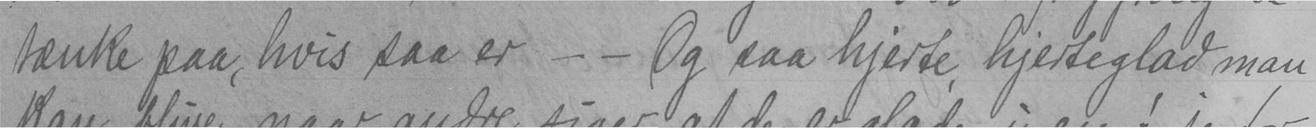tænke paa, hvis saa er - - Og saa hjerte, hjerteglad man
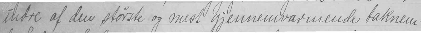indre af den største og mest gjennemvarmende taknem-
